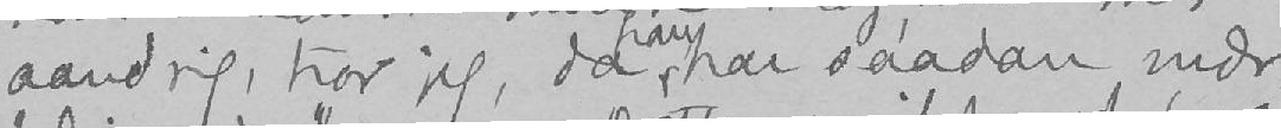aandrig, tror jeg, da han har saadan mær-
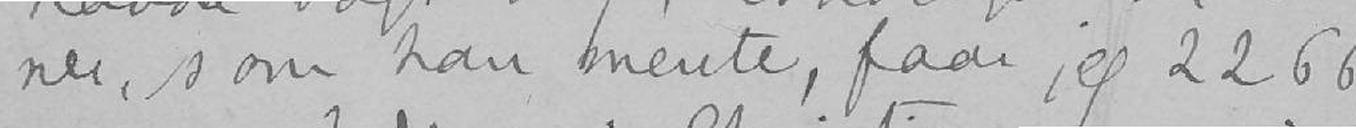ner, som han mente, faar jeg 2266,
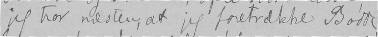jeg tror næsten, at jeg foretrækkede Bodde,
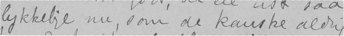lykkelige nu, som de kanske aldrig
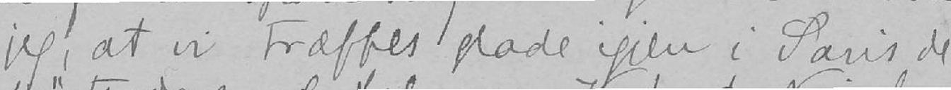jeg, at vi træffes glade igjen i Paris de
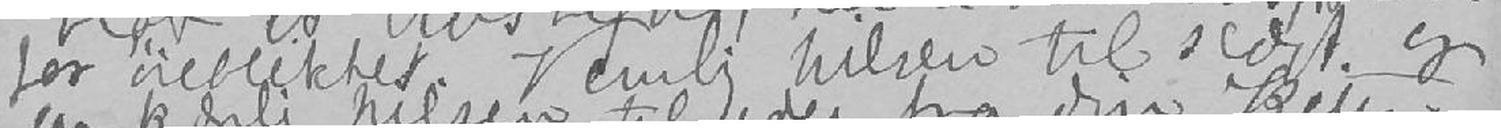for øieblikket. Venlig hilsen til slægt og
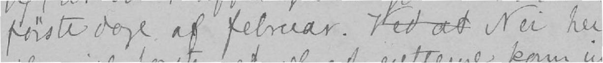første dage af februar. Ved at Nei her
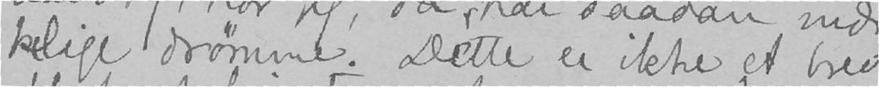kelige drømme. Dette er ikke et brev,
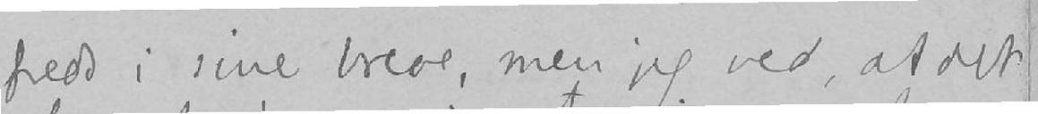i sine breve, men jeg ved, at det
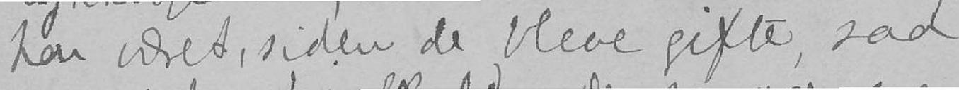har været, siden de bleve gifte, saa
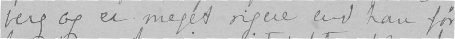berg og er meget rigere end han før
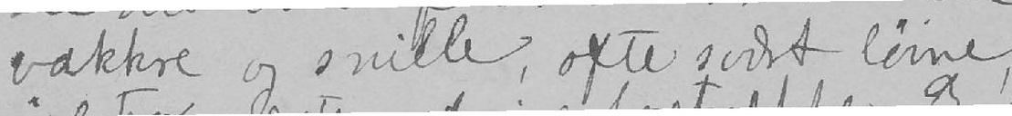vakkre og snille, ofte svært løine,
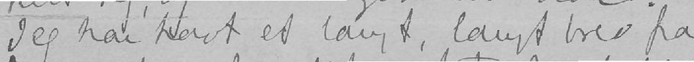Jeg har havt et langt, langt brev fra
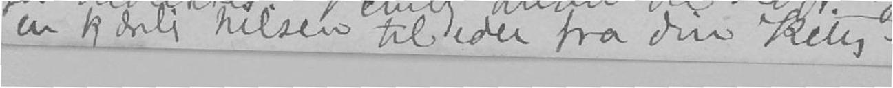en kjærli hilsen til eder fra din Kitty.
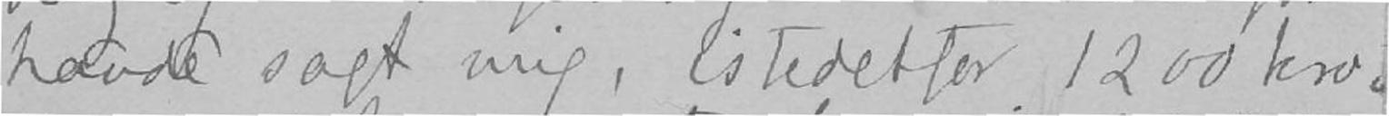havde sagt mig., istedetfor 1200 kro-
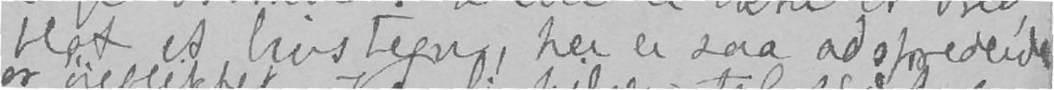blot et livstegn, her er saa adspredende
